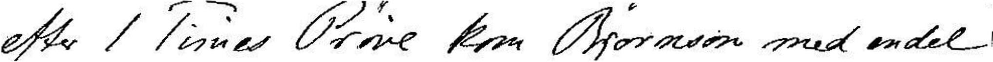efter 1 Times Prøve kom Bjørnson med endel
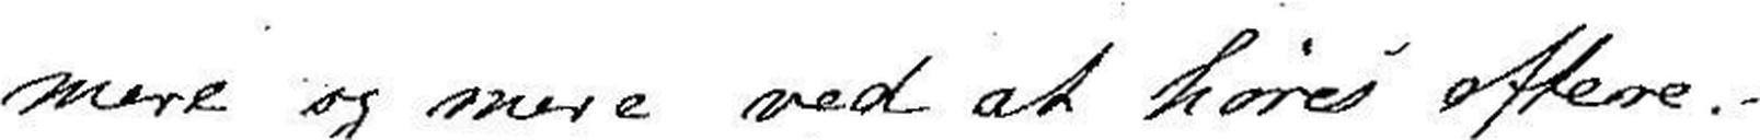mere og mere ved at høres oftere.-
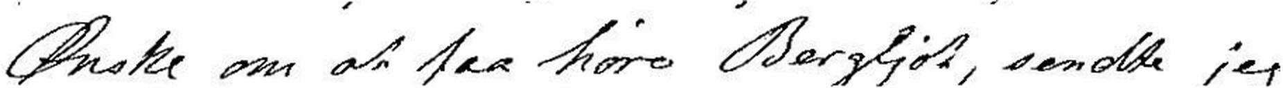Ønske om at faa høre Bergljot, sendte jeg
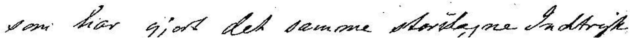som har gjort det samme storslagne Indtryk
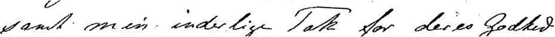samt min inderlige Tak for deres Godhed
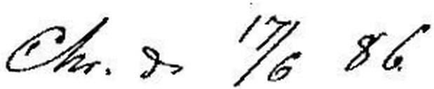Chr. d. 17/6 86
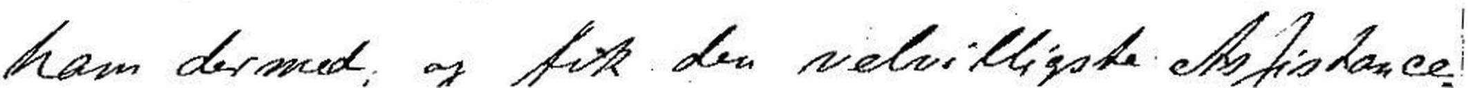ham dermed og fik den velvilligste Assistance;
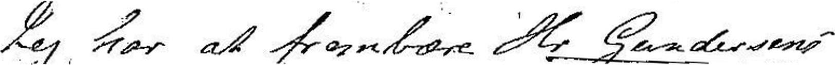Jeg har at frambære Hr Gundersens
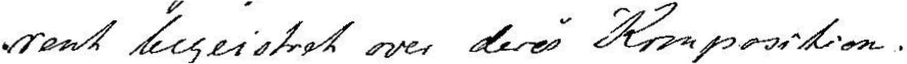rent begeistret over deres Komposition.
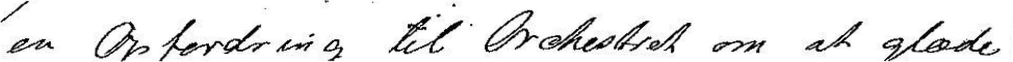en Opfordring til Orchertret om at glæde
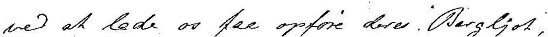ved at lade os faa opføre deres Bergljot,
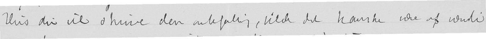Hvis du vil skrive den anbefaling, vilde det kanske være af værdi
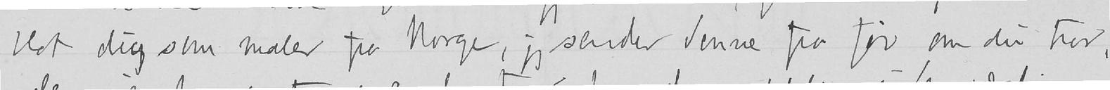blot dig som maler fra Norge, jeg sender denne fra før om du tror,
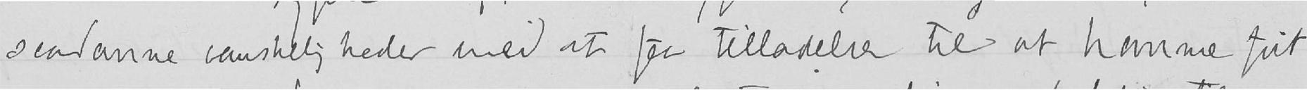saadanne vanskeligheder med at faa tilladelse til at komme frit
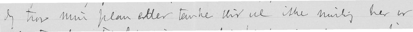Jeg tror min plan eller tanke blir vel ikke mulig her er
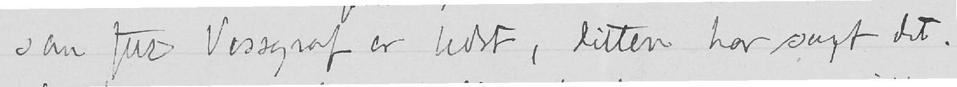som frk Vossgraf, er bedst, Ditten har sagt det.
